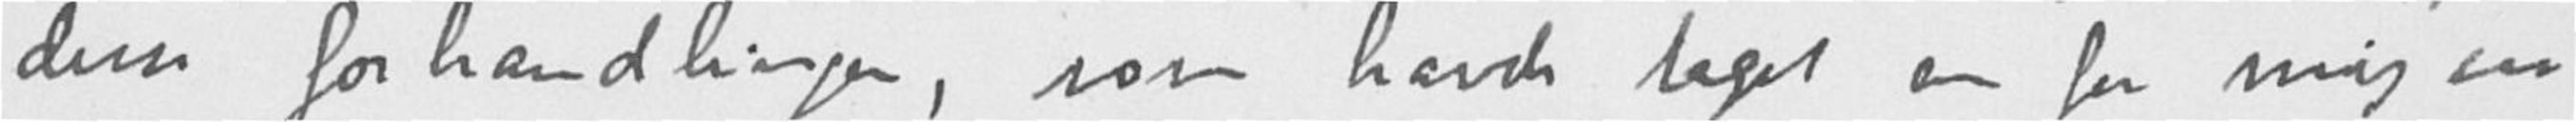disse forhandlinger, som havde taget en for mig saa
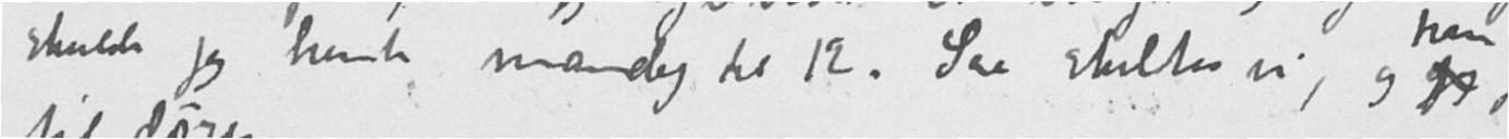skulde jeg hente mandag kl 12. Saa skiltes vi, og jeg
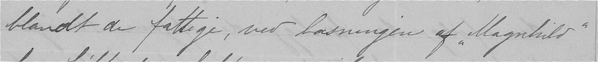blandt de fattige, ved læsningen af "Magnhild"
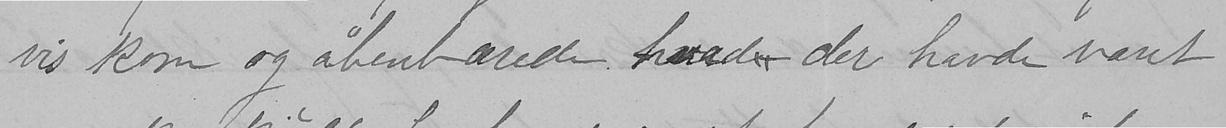vis kom og åbenbarede hvad der havde været
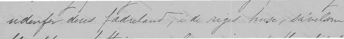udenfor deres fædreland, i de riges huse, såvelsom
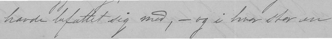havde befattet sig med, - og i hvor stor en
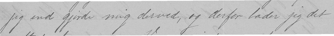jeg end gjorde mig derved, og derfor lader jeg det
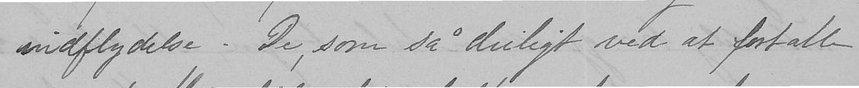indflydelse. De, som så deiligt ved at fortælle
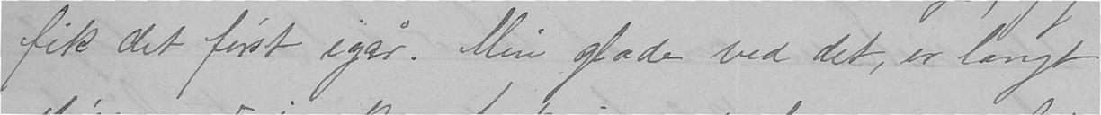fik det først igår. Min glæde ved det, er langt
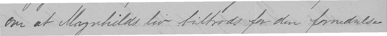om at Magnhilds liv tiltrods for den fornedrelse
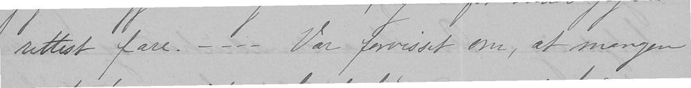rettest fare. - - - - Vær forvisset om, at mangen
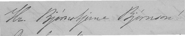Hr. Bjørnestjerne Bjørnson!
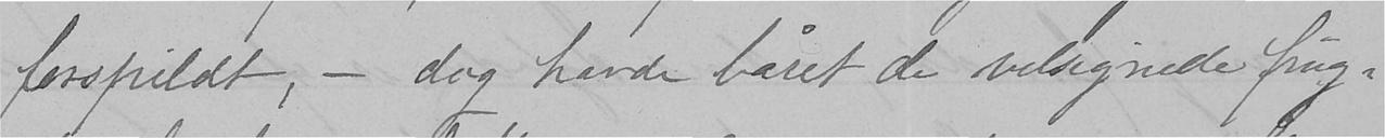forspildt, - dog havde båret de velsignede frug-
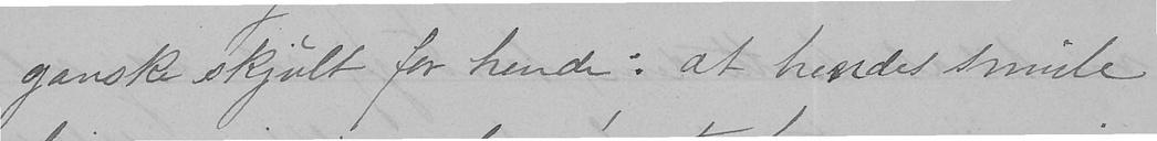ganske skjult for hende: at hendes smule
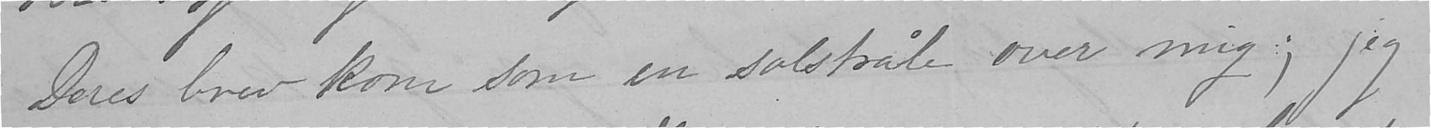Deres brev kom som en solstråle over mig; jeg
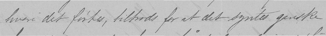hvori det førte, tiltrods for at det syntes ganske
